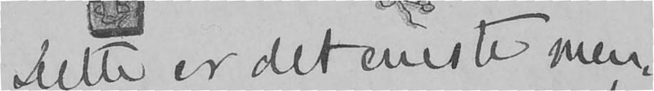Dette er det eneste men-
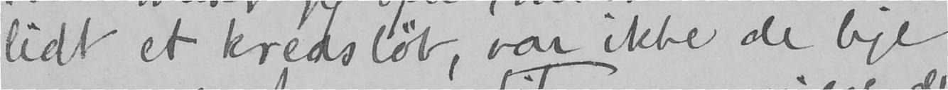lidt et kredsløb, var ikke de lige
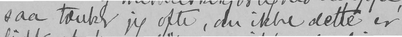saa tænker jeg ofte, du ikke dette er
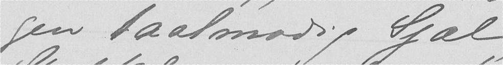gen taalmodig Sjæl,
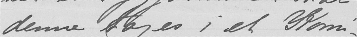denne tages i et Komi-
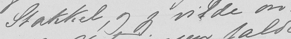Stakkel, og jeg vilde øn-
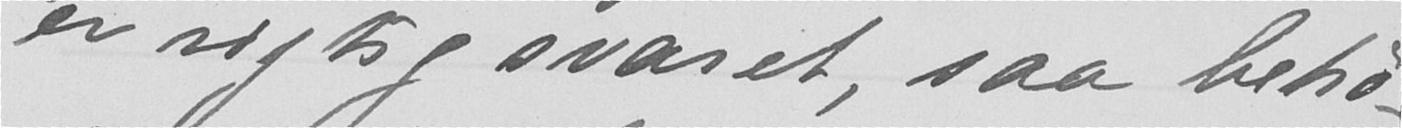er rigtig svaret, saa behø-
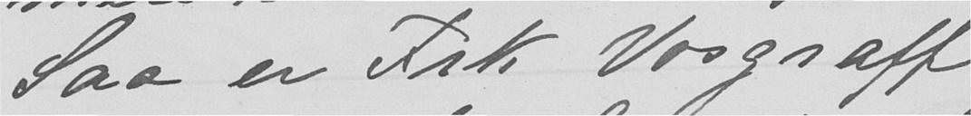Saa er Frk Vosgraff
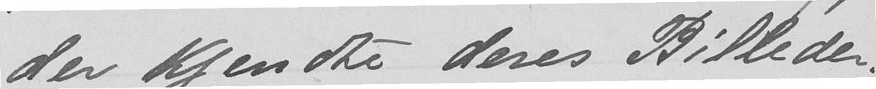der kjendte deres Billeder.
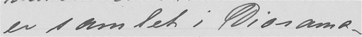er samlet i Diorama-
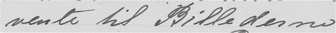vente til Billederne
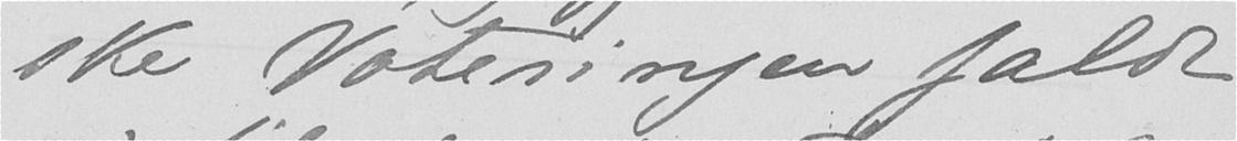ske Voteringen faldt
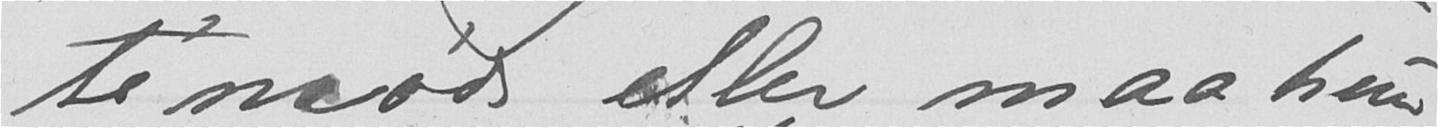témøde eller maa hun
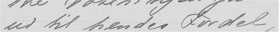ud til hendes Fordel,
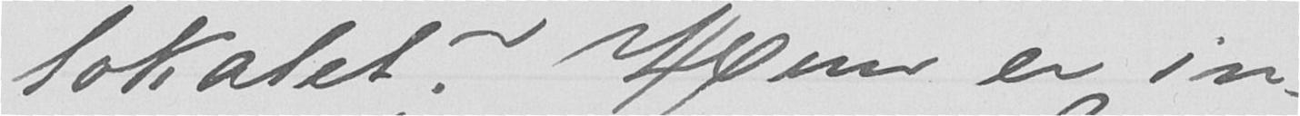lokalet? Hun er in-
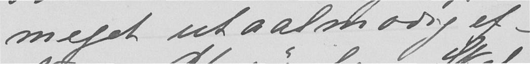meget utaalmodig ef-
